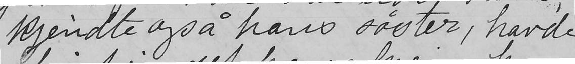kjendte også hans søster, havde
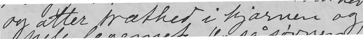og atter træthed i hjærnen og
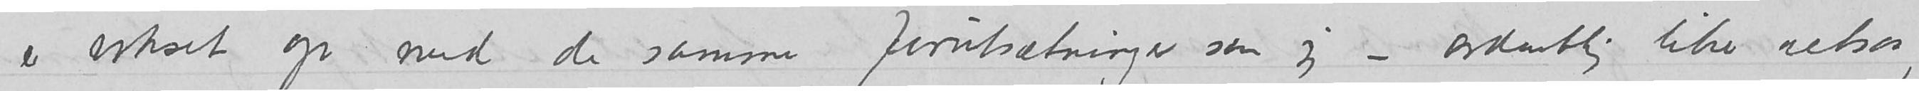mennesker som er vokset op med de samme forutsætninger som jeg – ordentlig like altsaa,
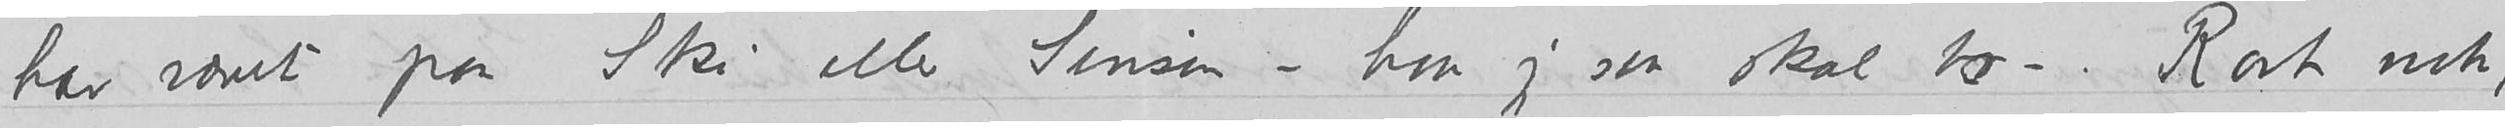har været på Ski eller Sinsen – hvor jeg saa skal bo –-. Rart nok,
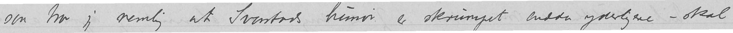saa tror jeg nemlig at Svarstads humør er skrumpet endda yderligere – skal
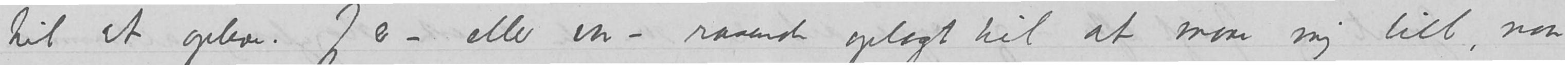til at opleve. Jeg er – eller var –- rasende oplagt til at more mig litt, naar
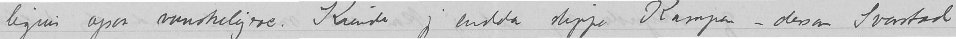ogsaa vanskeligere. Kunde jeg endda slippe Kampen – dersom Svarstad
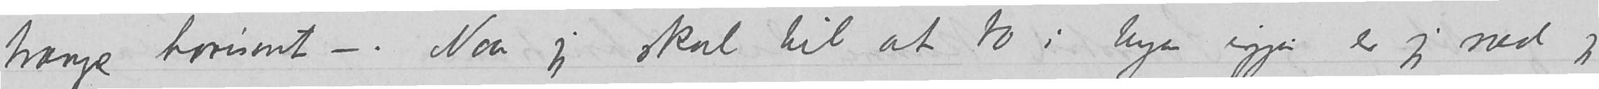trange horisont –. Naar jeg skal til at bo i byen igjen er jeg ræd jeg
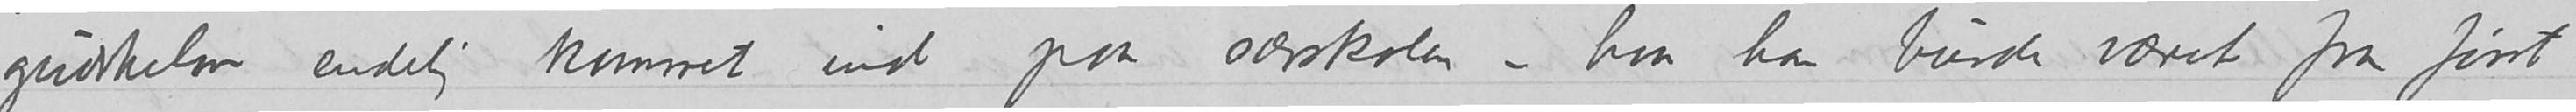gudskelov endelig kommet ind paa særskolen – hvor han burde været fra først
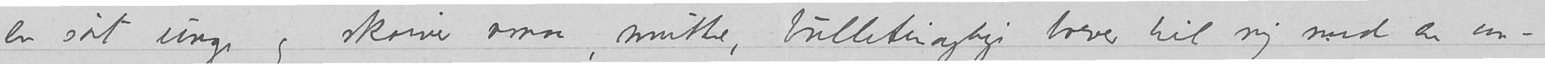en søt unge og skriver smaa, smukke, bulletinagtige brever til mig med en an-
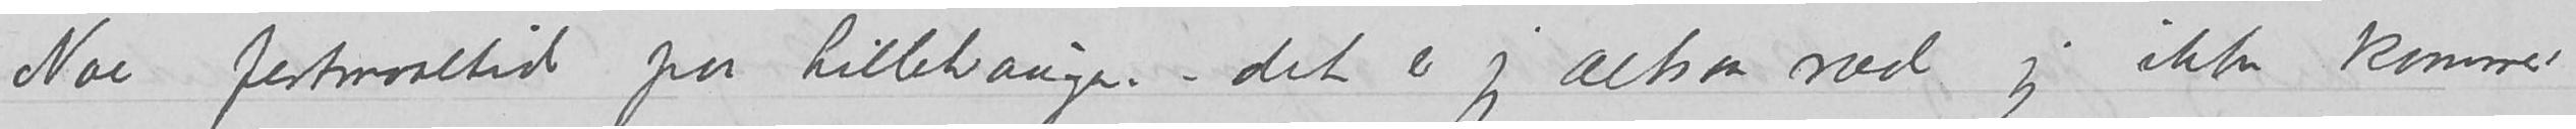Noe festmaaltid paa Lillehaugen – det er jeg altsaa ræd jeg ikke kommer
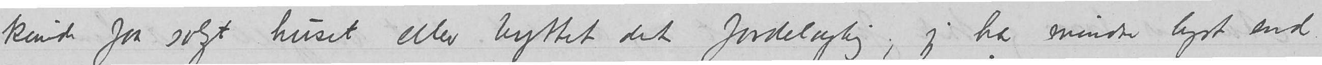kunde faa solgt huset eller byttet det fordelagtig;  jeg har mindre lyst end
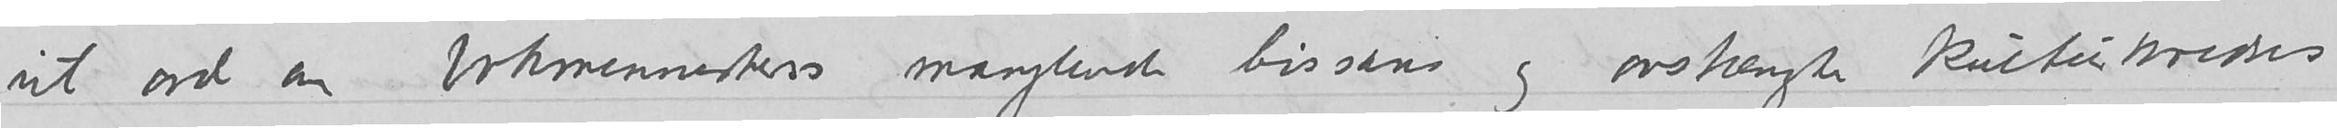ut ord om bokmenneskers manglende livssans og avstængte kulturkredser
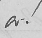av!
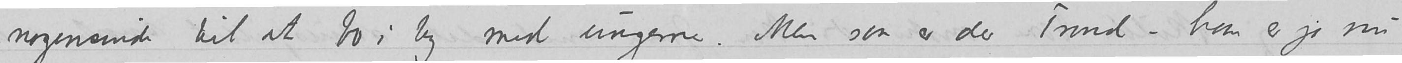nogensinde til at bo i by med ungerne. Men saa er der Trond – han er jo nu
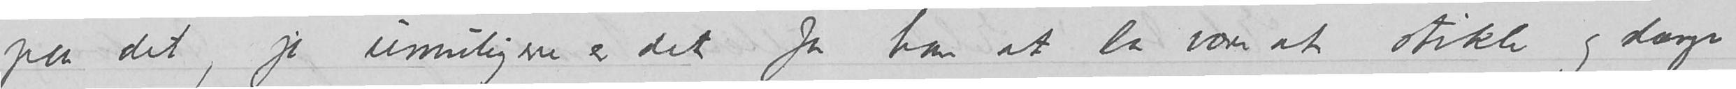paa det, jo umuligere er det for han at la være at stikke og slænge ut ord om bokmenneskers manglende livssans og avstængte kulturkredse<r>s trange horisont –-.  Naar jeg skal til at bo i byen igjen er jeg ræd jeg
